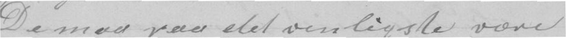De maa paa det venligste være
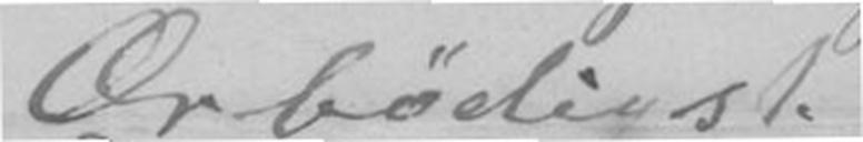Ærbødigst
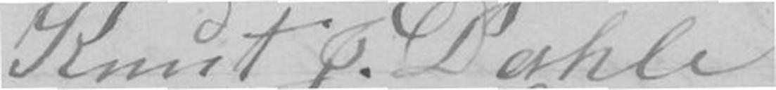Knut J. Dahle
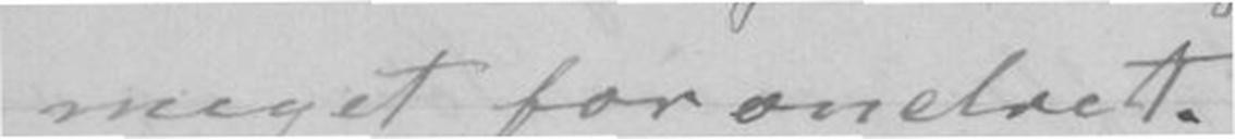meget forandret.
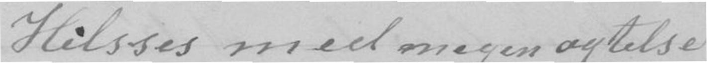Hilsses med megen agtelse
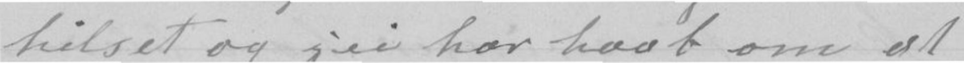hilset og jei har haab om at
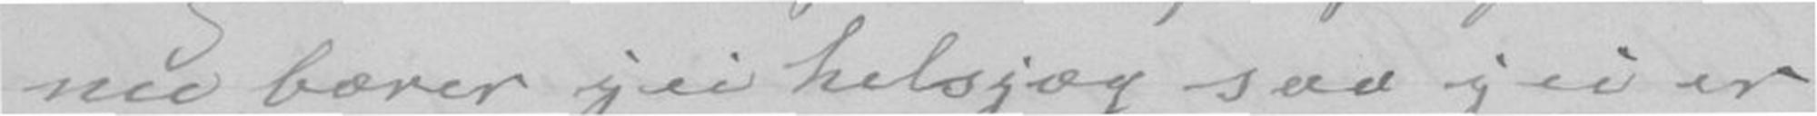nu bærer jei helsjæg saa jei er
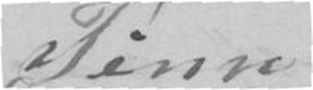Tinn
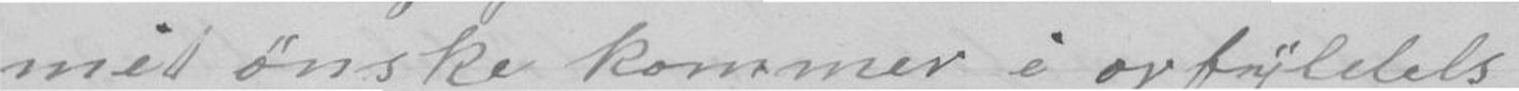mit ønske kommer i opfyldels
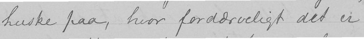huske paa, hvor fordærveligt det er
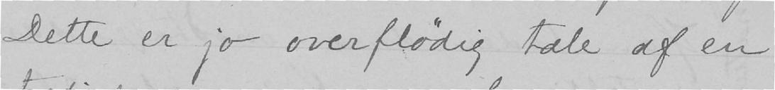Dette er jo overflødig tale af en
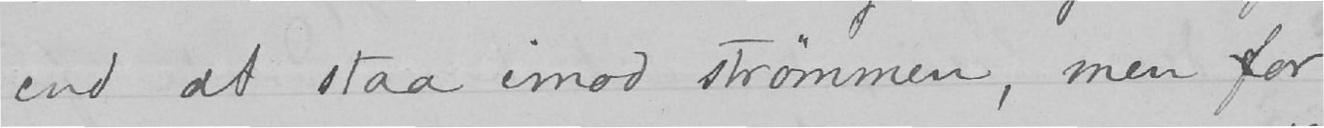end at staa imod strømmen, men for
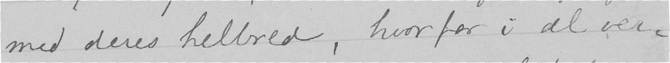med deres helbred, hvorfor i al ver-
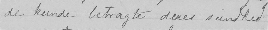de kunde betragte deres sundhed
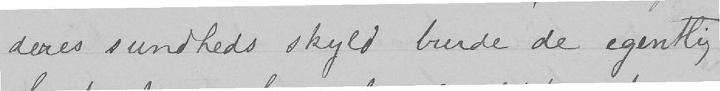deres sundheds skyld burde de egentlig
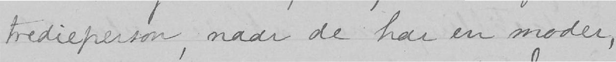tredieperson, naar de har en moder,
